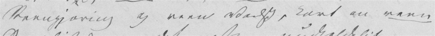Reengjøring og reen Vadsk, kort en reen
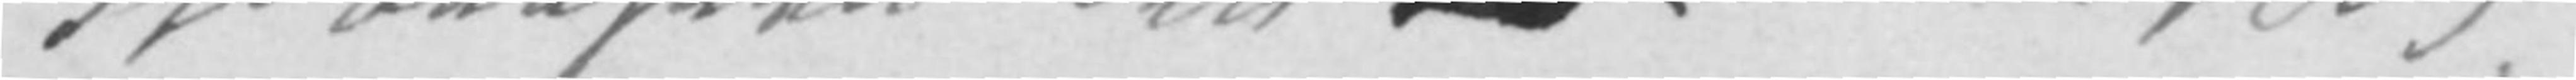Kjøbenhavn den 20de Mai 1853.
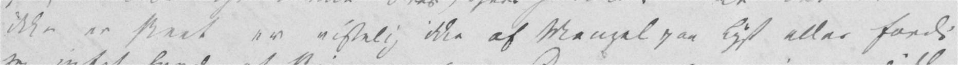ikke er skeet er visselig ikke af Mangel paa Lyst eller fordi
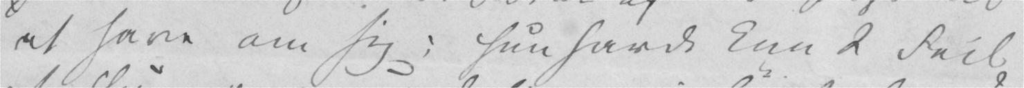at have om sig; hun havde kun 2 Feil
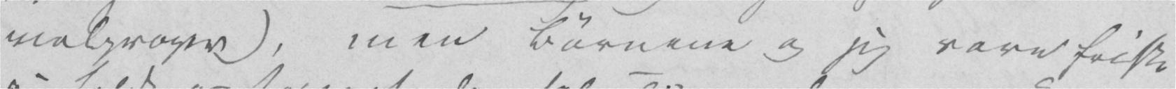malproper), men Børnene og jeg vare friske
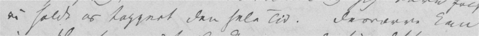vi holdt os tappert den hele Tid. Desværre kan
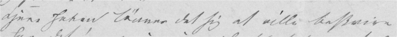Kjere Johan lønner det sig at ville beskrive
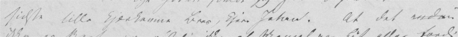sidste lille kjærkomne Brev, kjere Johan. At det endnu
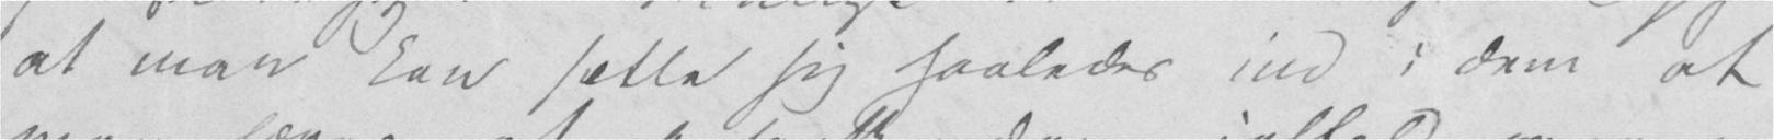at man kan sætte sig saaledes ind i dem at
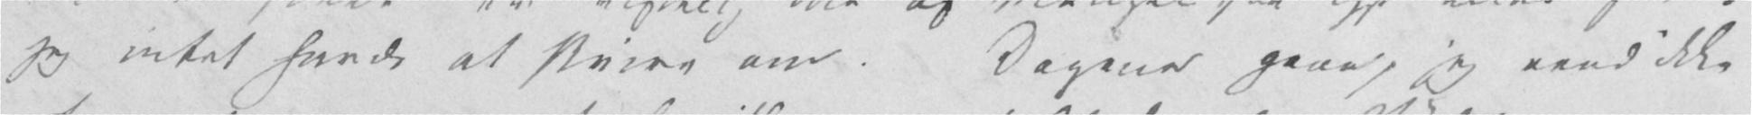jeg intet havde at skrive om. Dagene gaae, jeg veed ikke
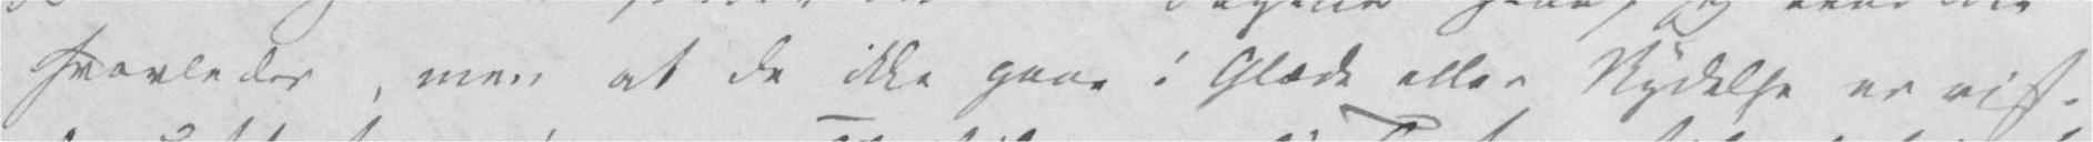hvorledes, men at de ikke gaae i Glæde eller Nydelse er vist,
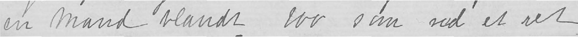en Mand blandt 100 som ved et ret
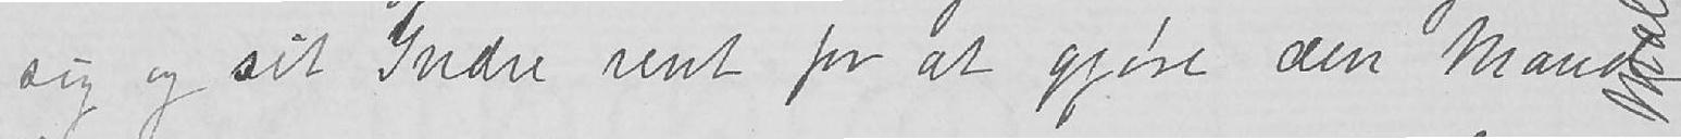sig og sit Indre rent for at gjøre den Mand
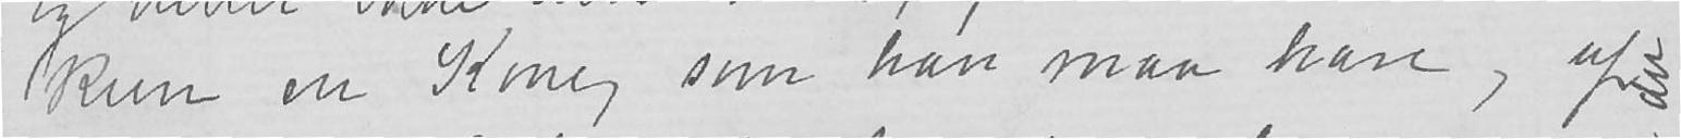kun en Kone, som han maa have, uf
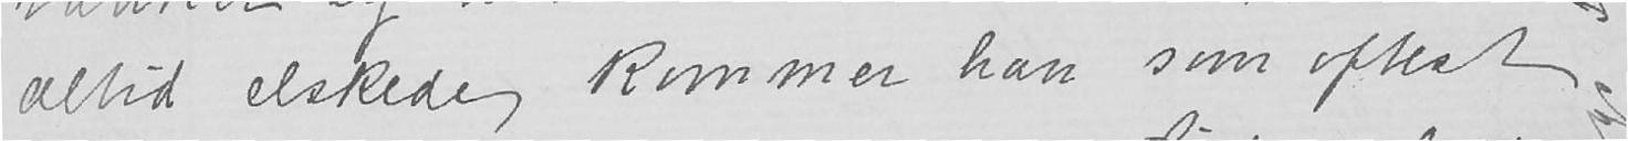altid elskede, kommer han som oftest
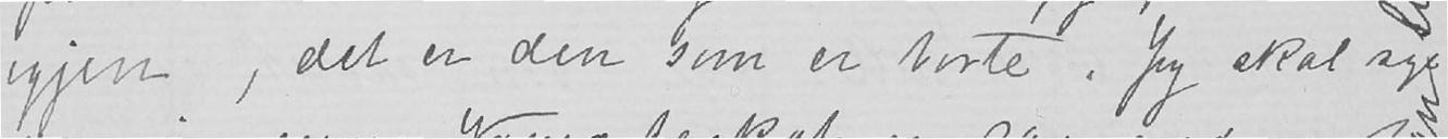igjen, det er den som er borte. Jeg skal sige
